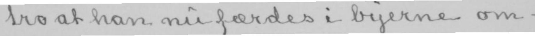tro at han nu færdes i byerne om-
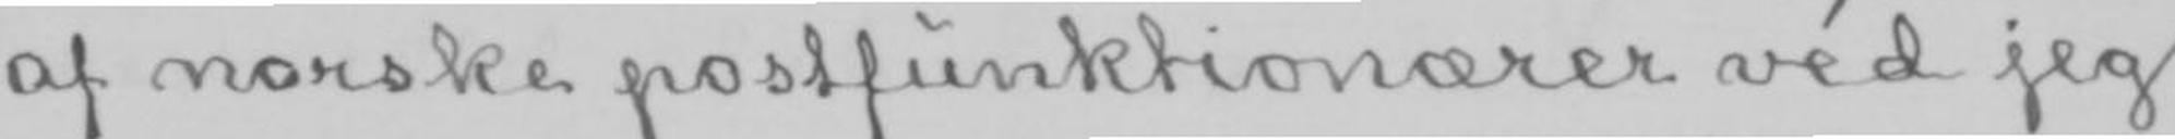af norske postfunktionærer véd jeg
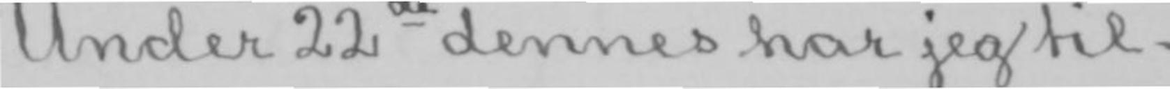Under 22de dennes har jeg til-
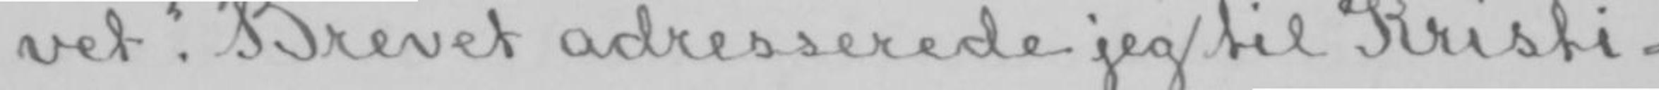vet». Brevet adresserede jeg til Kristi-
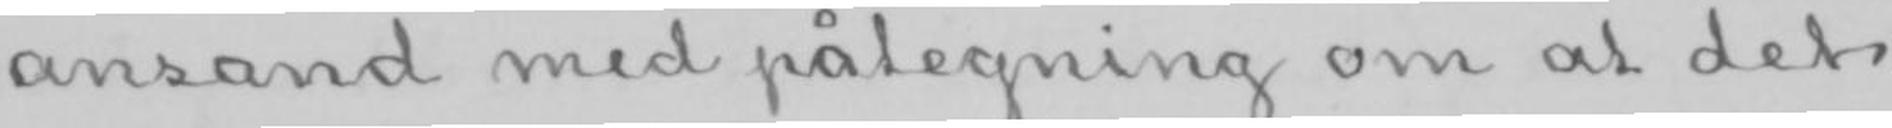ansand med påtegning om at det
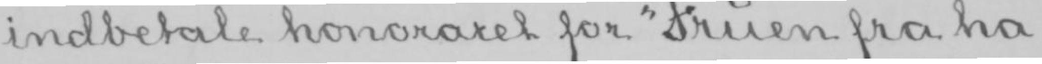indbetale honoraret for «Fruen fra ha-
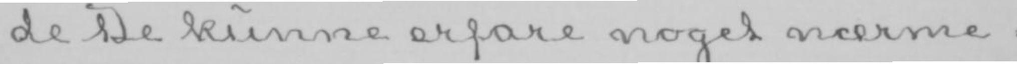de De kunne erfare noget nærme-
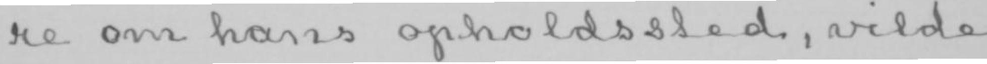re om hans opholdssted, vilde
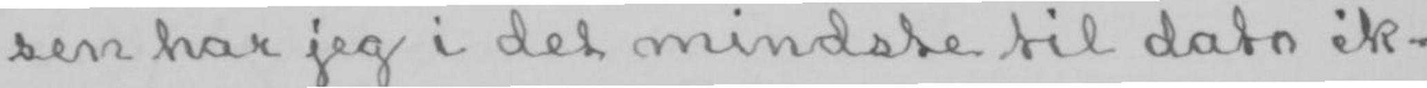sen har jeg i det mindste til dato ik-
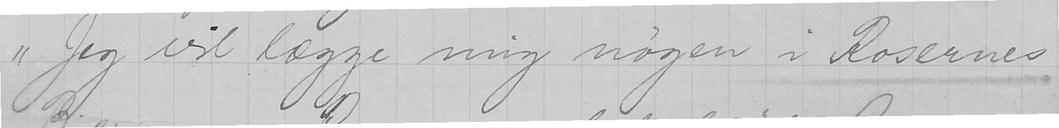"Jeg vil lægge mig nøgen i Rosernes
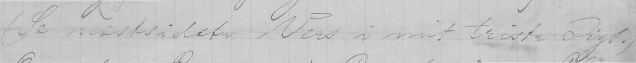(Se næstsidste Vers i mit triste Digt.)
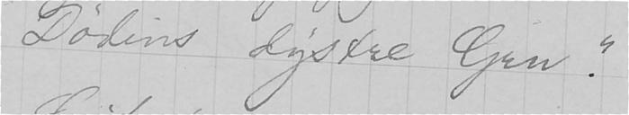Dødens dystre Gru".
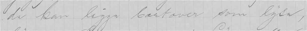de kan ligge bortover som lyse,
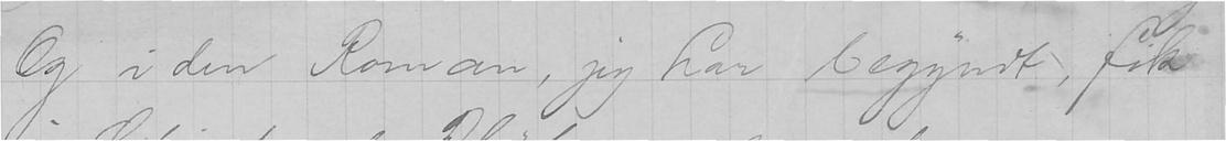Og i den Roman, jeg har begyndt, fik
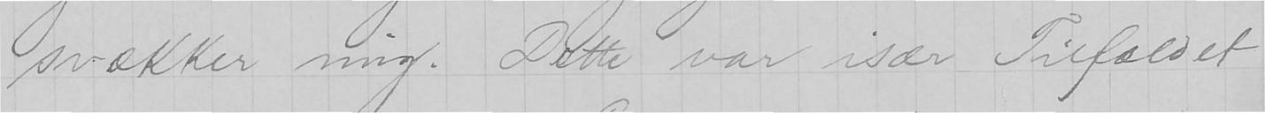svækker mig. Dette var især Tilfældet
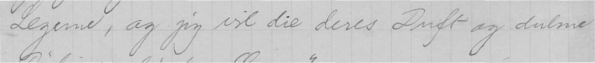Legeme, og jeg vil die deres duft og dulme
